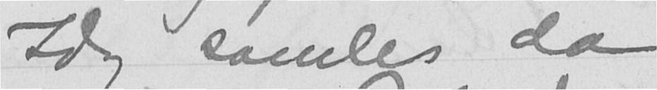Idag samles da
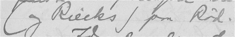(og Riecks) paa Rød.
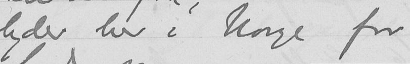lyder her i Norge for
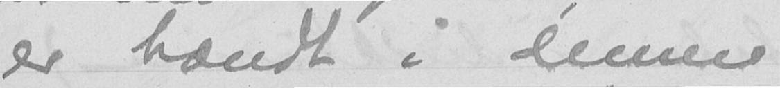er hændt i denne
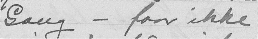Gang - faar ikke
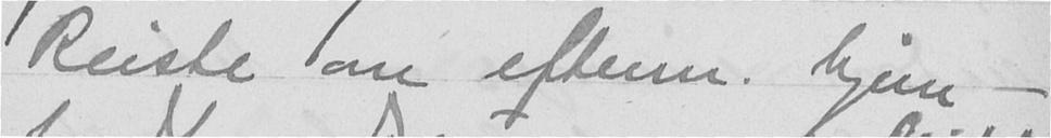Reiste om efterm. hjem -
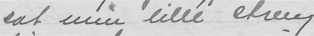sat min lille streng
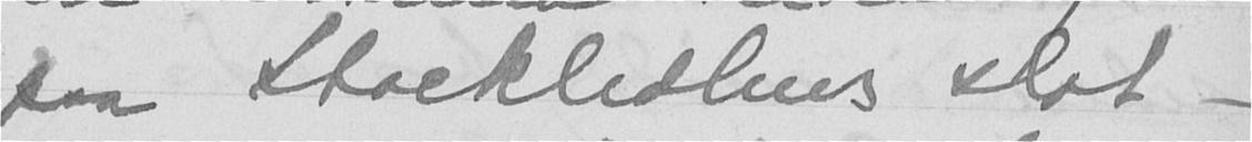paa Stockholms slot -
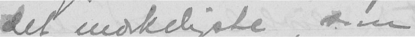det mærkeligste, som
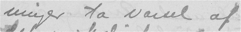ninger ta varsel af
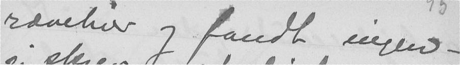rævehier og fandt ingen.
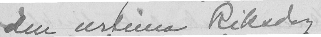den urtima Riksdag
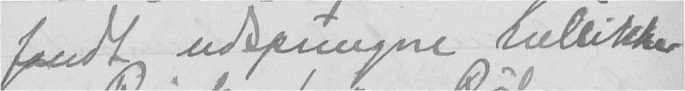fandt udsprungne nellikker
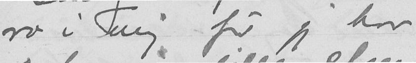ro i mig før jeg har
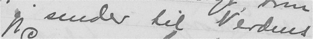jeg sender til Verdens
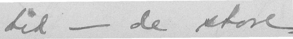tid - de store
